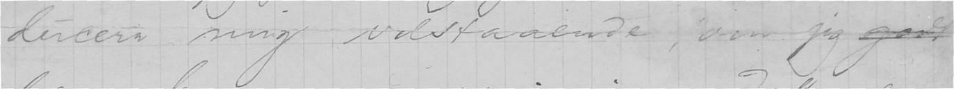ducere mig velstaaende, om jeg godt
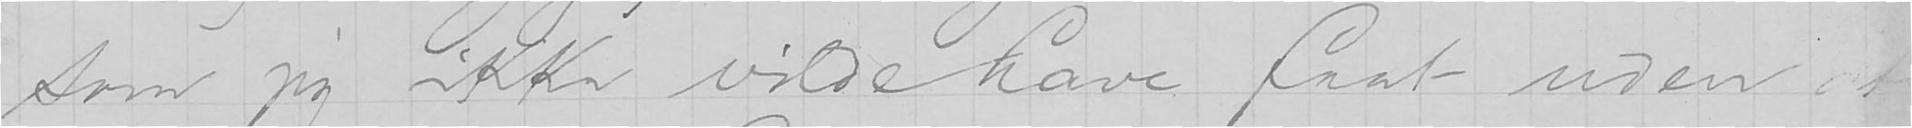som jeg ikke vilde have faat uden at
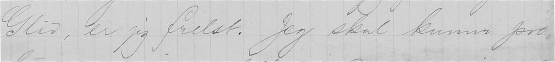Glid, er jeg frelst. Jeg skal kunne pro-
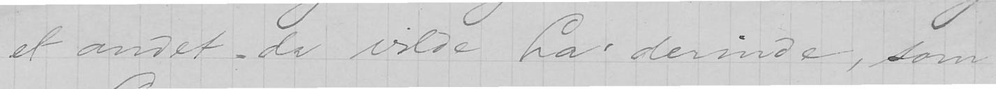et andet de vilde ha derinde, som
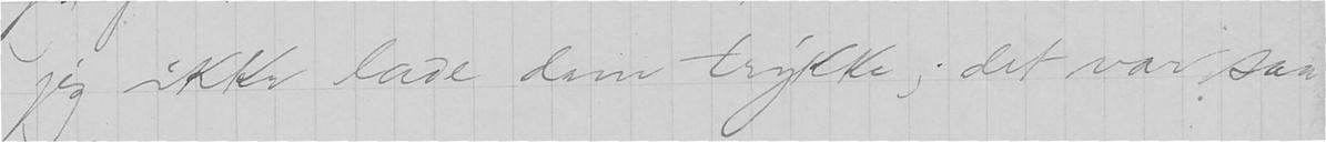jeg ikke lade dem trykke; det var saa
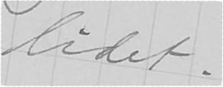lidet.
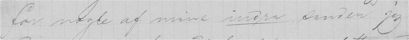for nogle af mine indre, sender jeg
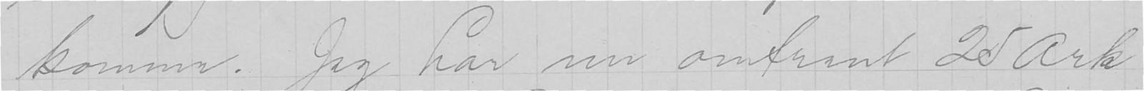komme. Jeg har nu omtrent 25 Ark
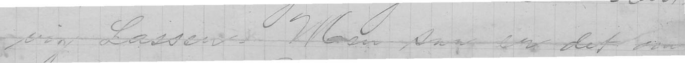vig Lassen. Men saa er det om
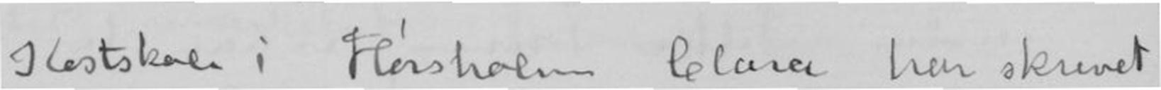Kostskole i Hersholm, Clara har skrevet
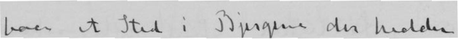bor et Sted i Bjergene der hedder
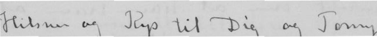Hilsner og Kys til Dig og Tonny
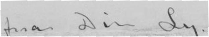fra Din Ly.
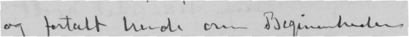og fortalt hende om Begivenheden.
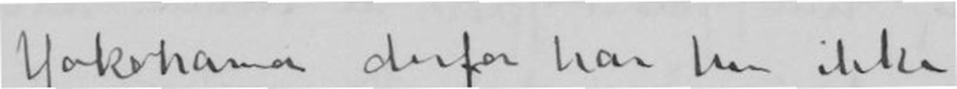i Yokohama derfor har hund ikke
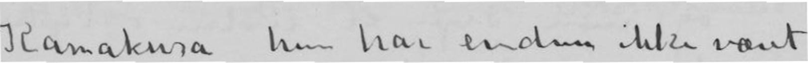Kamakura, hun har endun ikke været
